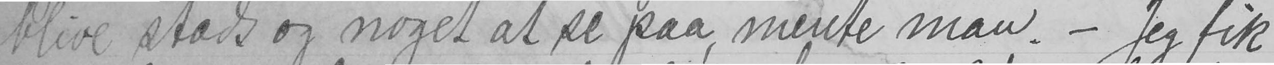blive stads og noget at se paa, mente man. - Jeg fik
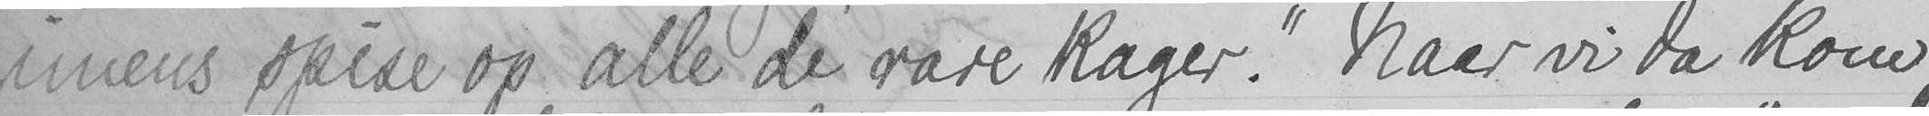imens spise op alle de rare kager." Naar vi da kom
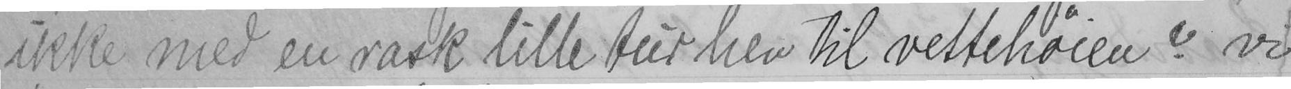ikke med en rask lille tur hen til vettehøien? vi
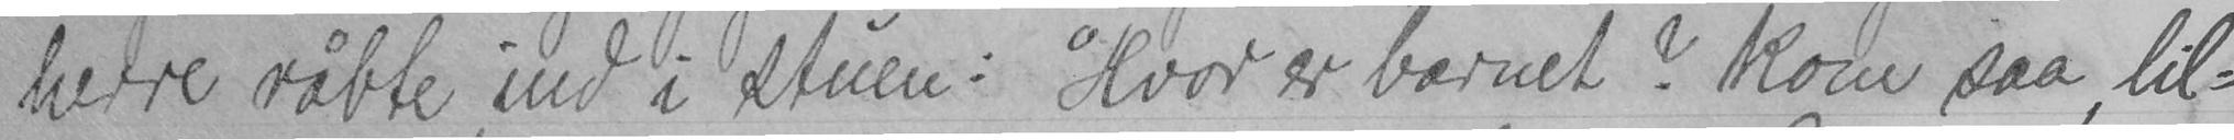herre råbte ind i stuen: Hvor er barnet? Kom saa, lil-
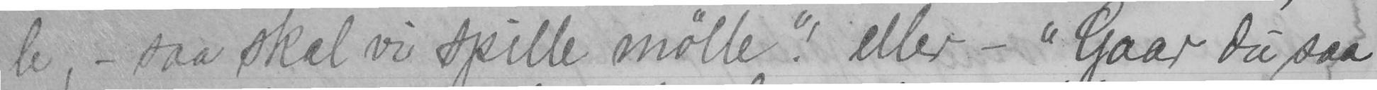le, - saa skal vi spille mølle"! eller - "Gaar du saa
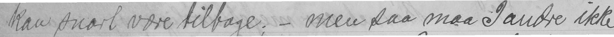kan snart være tilbage; - men saa maa I andre ikke
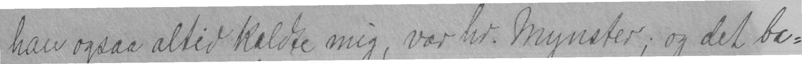han ogsaa altid kaldte mig, var hr. Mynster; og det ba-
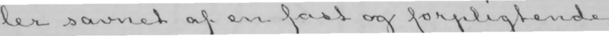ler savnet af en fast og forpligtende
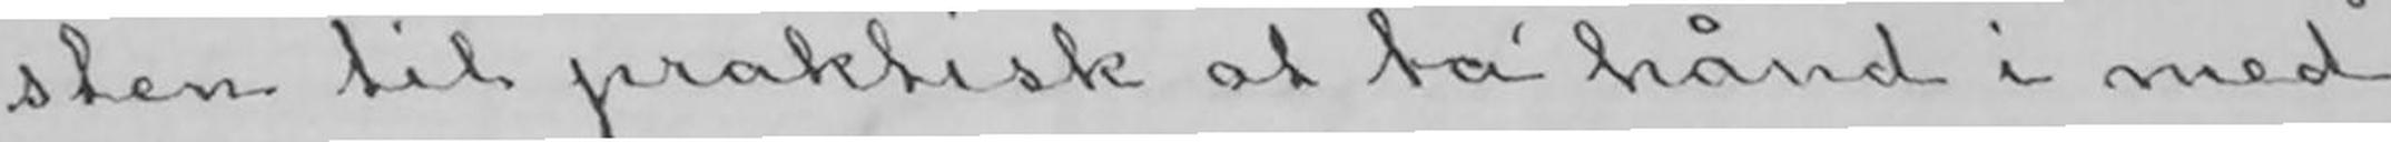sten til praktisk at ta´ hånd i med
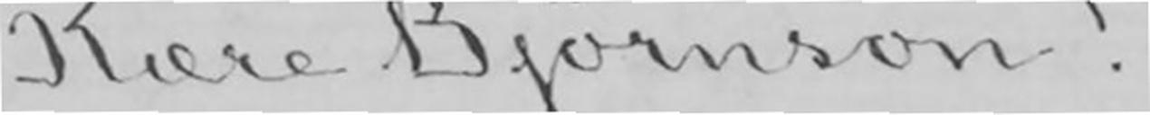Kære Bjørnson!
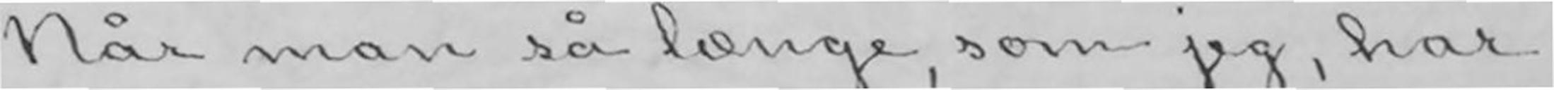Når man så længe, som jeg, har
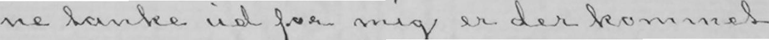ne tanke ud for mig er der kommet
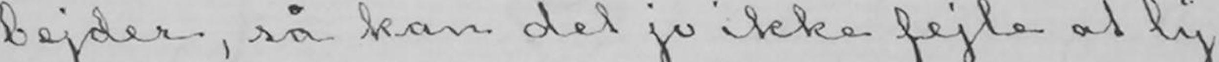bejder, så kan det jo ikke fejle at ly-
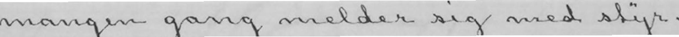mangen gang melder sig med styr-
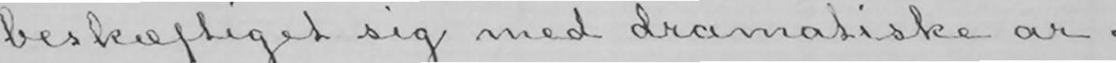beskæftiget sig med dramatiske ar-
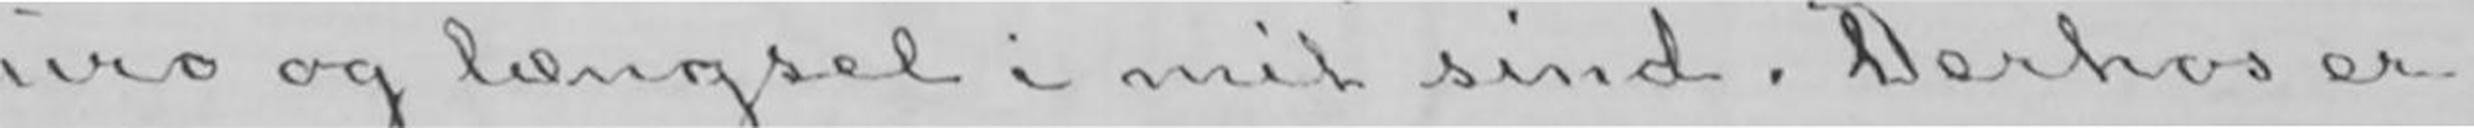uro og længsel i mit sind. Derhos er
